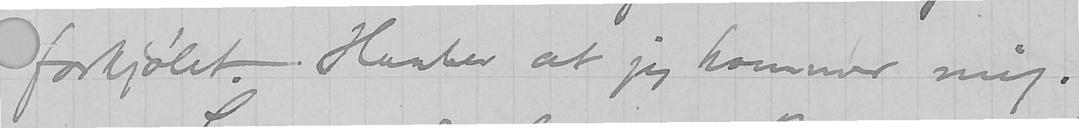forkjølet. Haaber at jeg kommer mig.
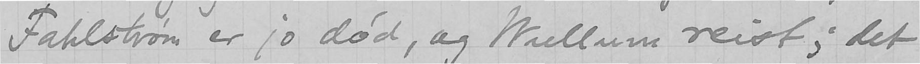Fahlstrøm er jo død, og Wullum reist; det
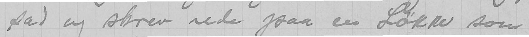sad og skrev ude paa en Løkke som
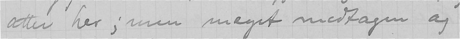atter her; men meget medtagen og
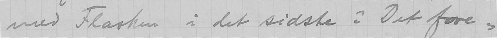med Flasken i det sidste? Det fore-
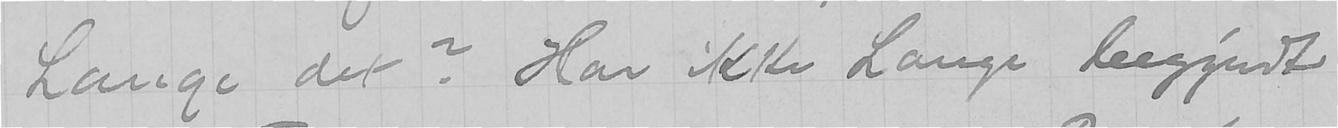Lange det? Har ikke Lange begyndt
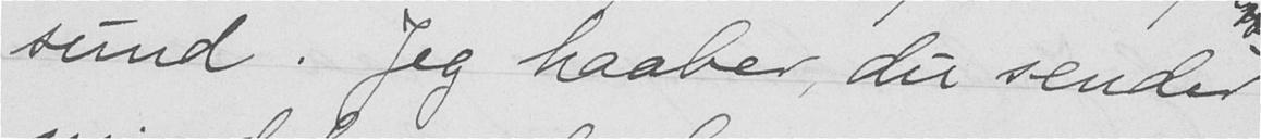sund. Jeg haaber, du sender
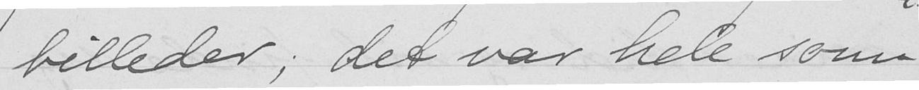billeder; det var hele som-
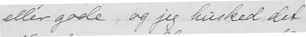eller gode, og jeg husked det
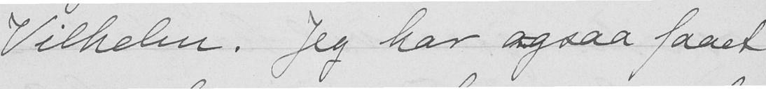Vilhelm. Jeg har ogsaa faaet
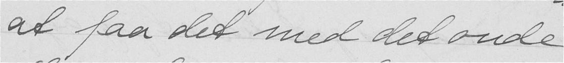at faa det med det onde
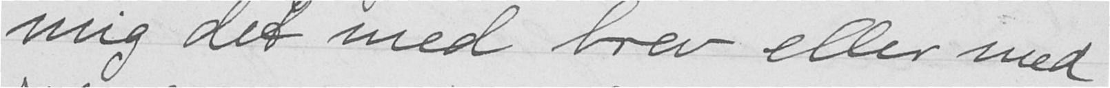mig det med brev eller med
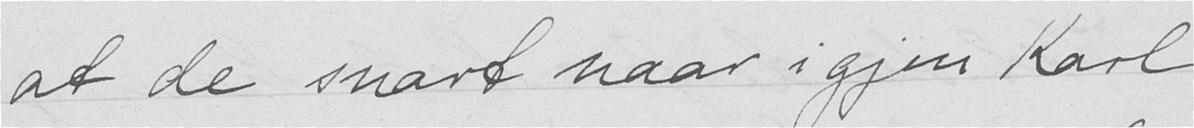at de snart naar igjen Karl
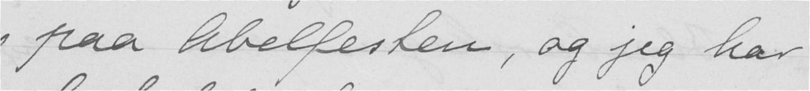paa Abelfesten, og jeg har
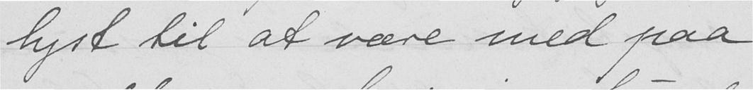lyst til at være med paa
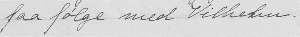faa følge med Vilhelm.
